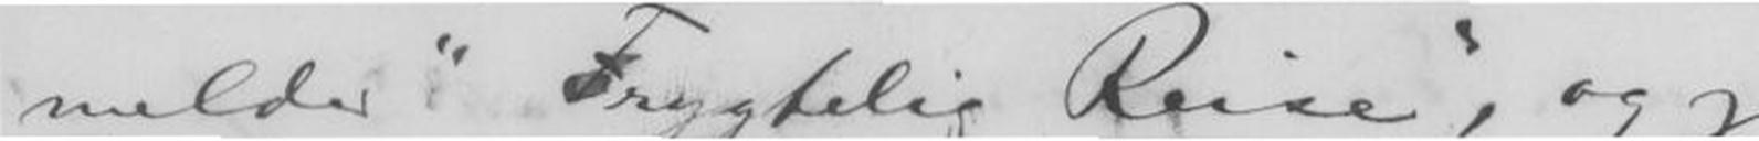melder "Frygtelig Reise", og jeg
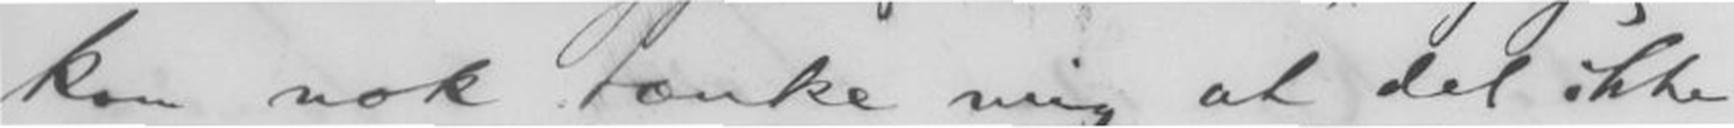kan nok tænke mig at det ikke
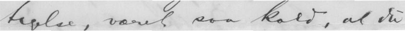tagelse, været saa kold, at Du
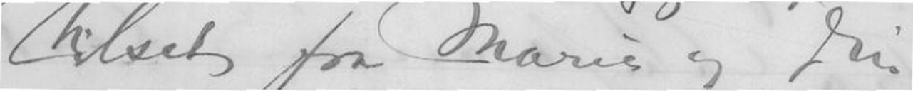hilset fra Marie og Din
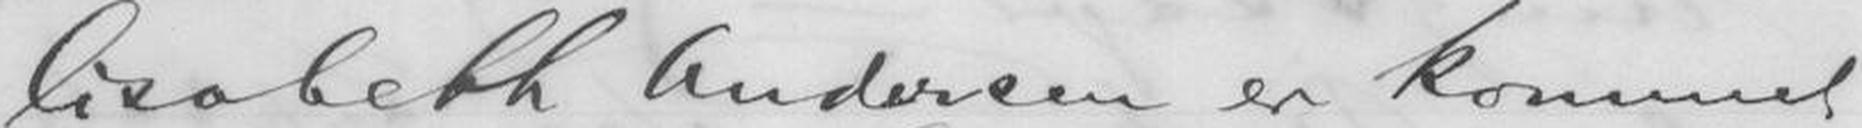lisabeth Andersen er kommet
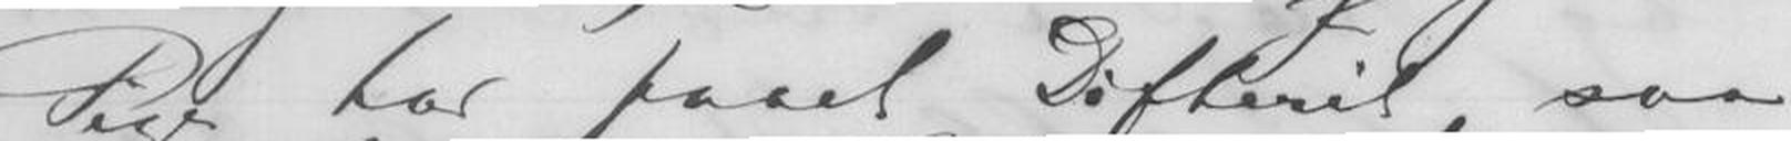Pige har faaet Difterit, saa
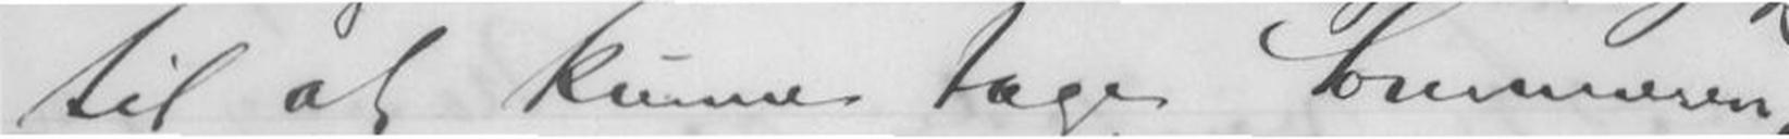til at kunne tage Sommeren,
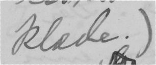klæde.
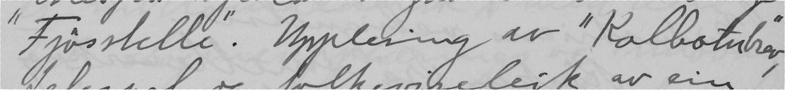"Fjösstelle". Upplesing av "Kolbotnbrev",
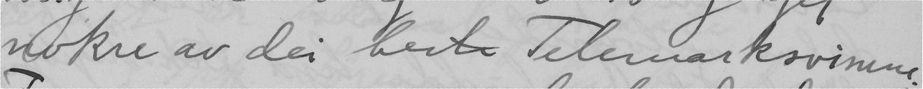nokre av dei beste Telemarksvisene.
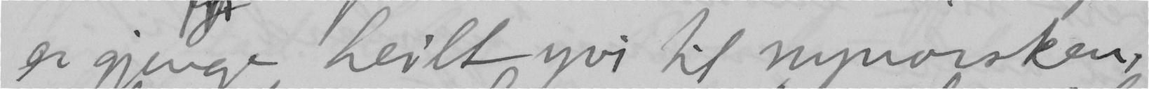er gjenge heilt yvi til nynorsken,
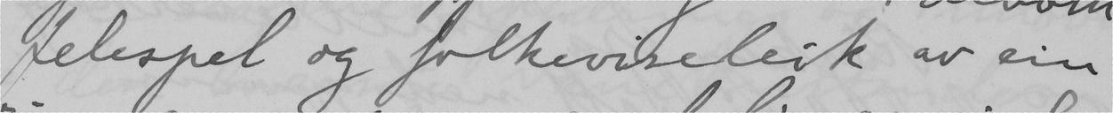felespel og folkeviseleik av ein
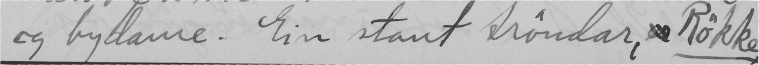og bydame. Ein staut tröndar, Rökke,
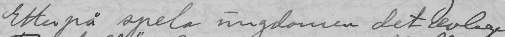Etterpå spela ungdomen det levlege
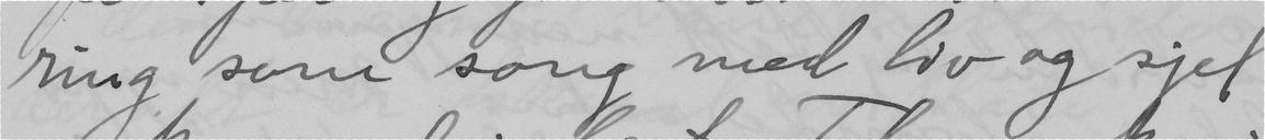ring som sang med liv og sjel
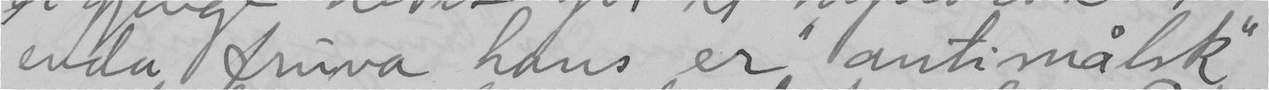enda fruva hans er "antimålsk"
